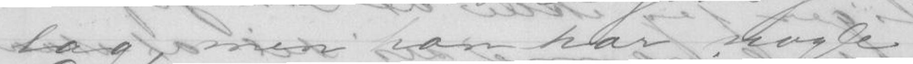lag, men han har nogle
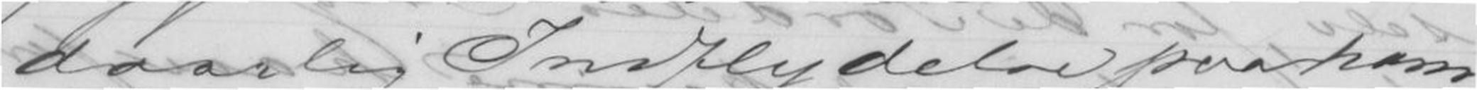daarlig Indflydelse paa ham.
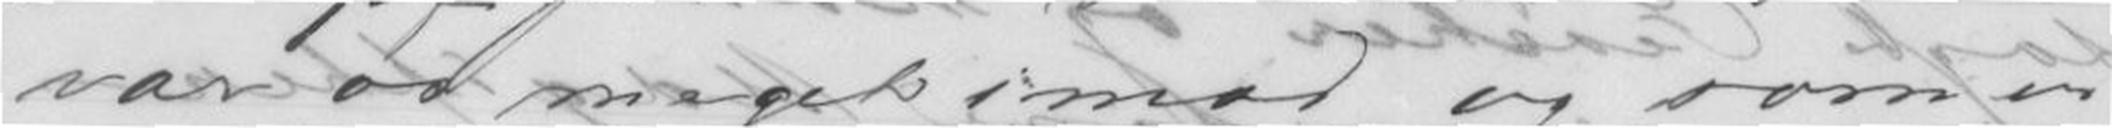var os meget imod, og som vi
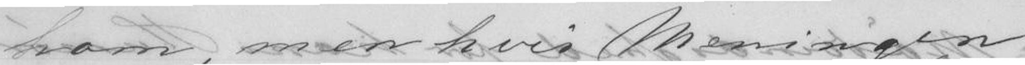ham, men hvis Meningen
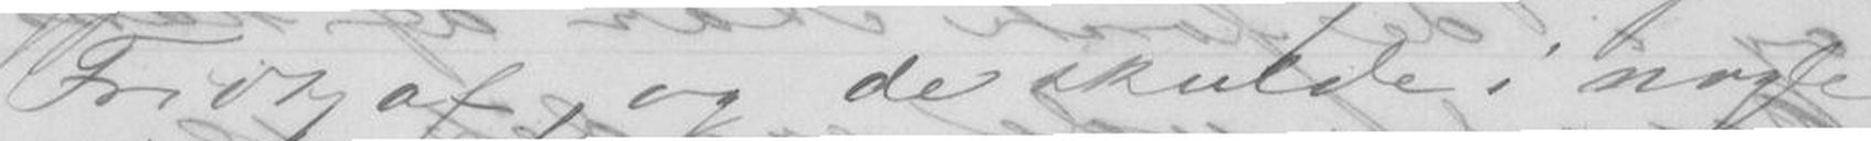Fridtjof, og de skulde i nogle
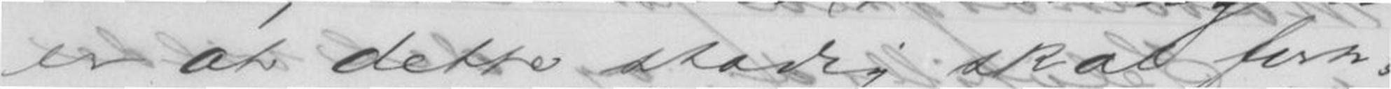er at dette stadig skal fort-
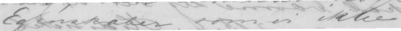Egenskaber, som vi ikke
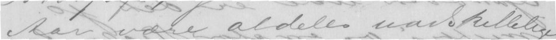Aar være aldeles uadskillelige,
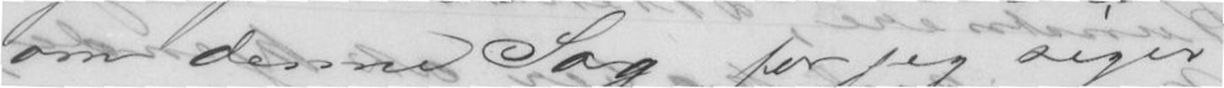om denne Sag, for jeg siger
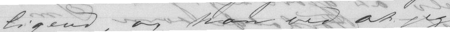ligeud, og han ved at jeg
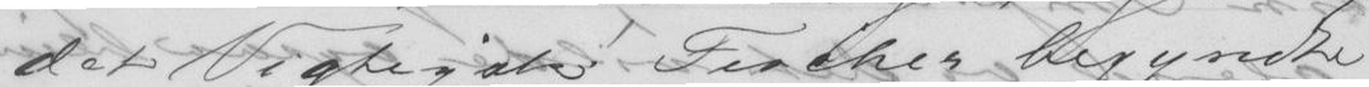det Vigtigste! Fischer begyndte
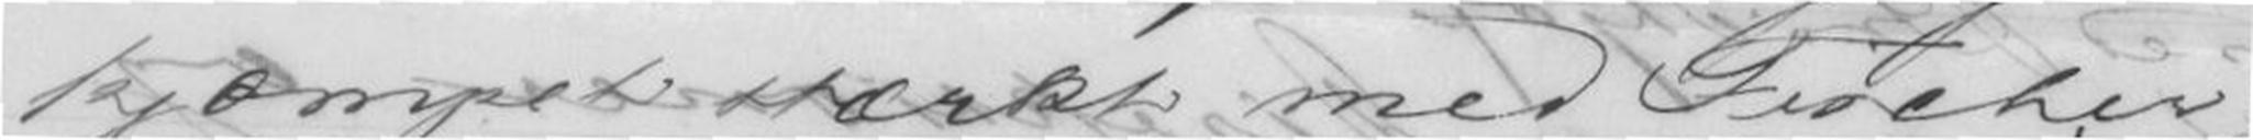kjæmpet stærkt med Fischer
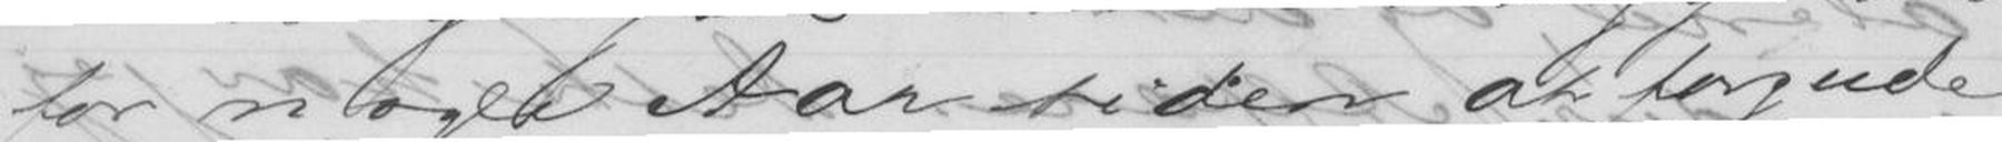for nogle Aar siden at forgude
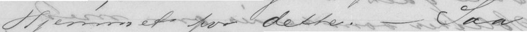Hjemmet for dette.- Saa
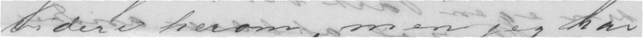videre herom, men jeg har
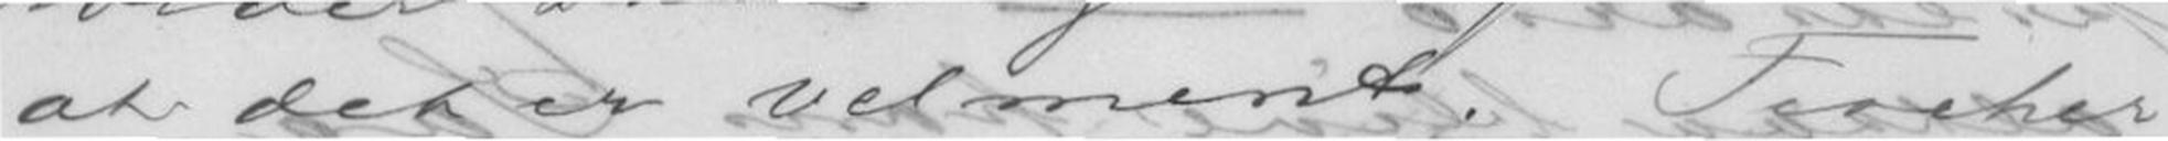at det er velment. Fischer
